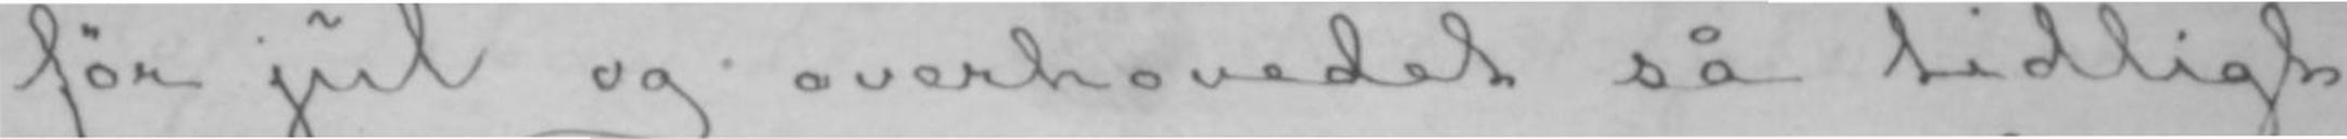før jul og overhovedet så tidligt
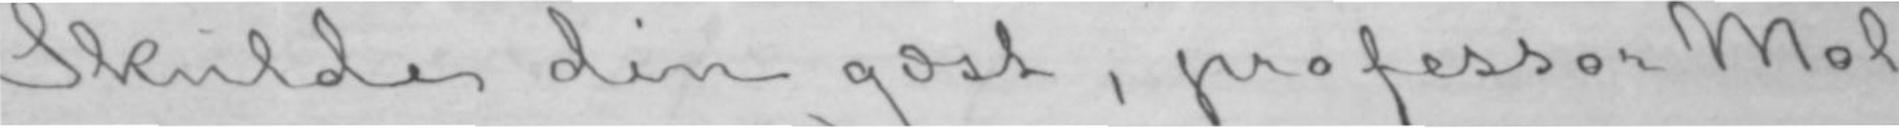Skulde din gæst, professor Mol-
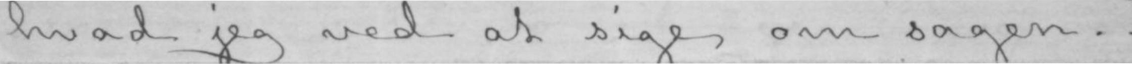hvad jeg ved at sige om sagen.-
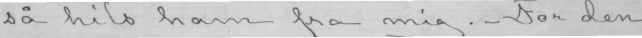så hils ham fra mig.- For den
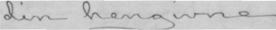din hengivne
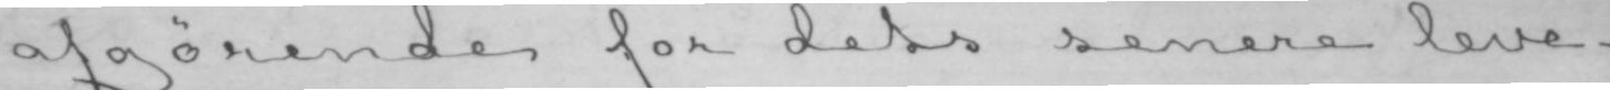afgørende for dets senere leve-
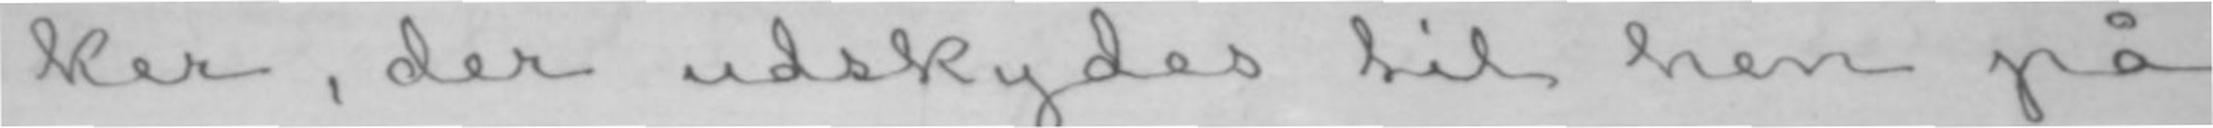ker, der udskydes til hen på
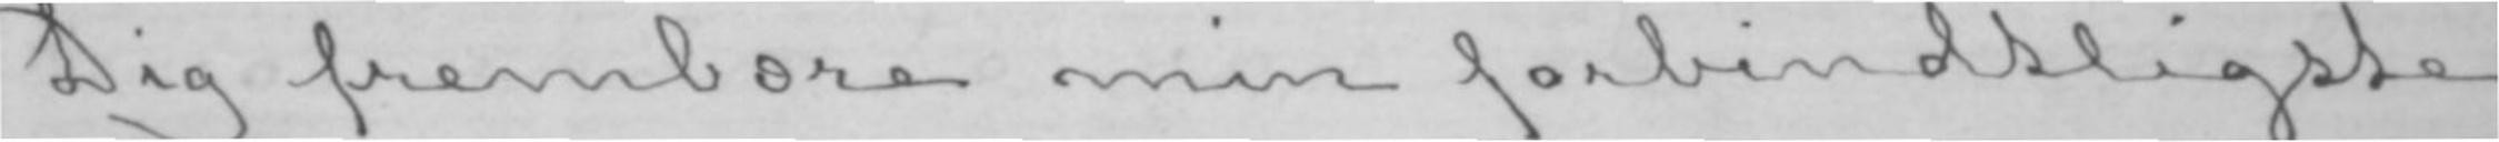Dig frembære min forbindtligste
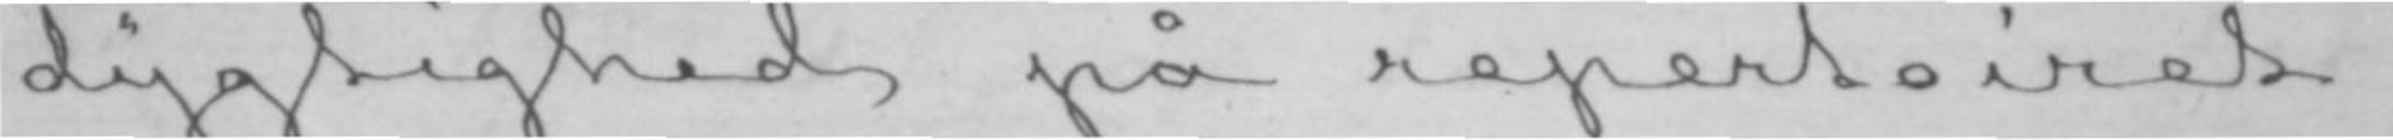dygtighed på repertoiret.
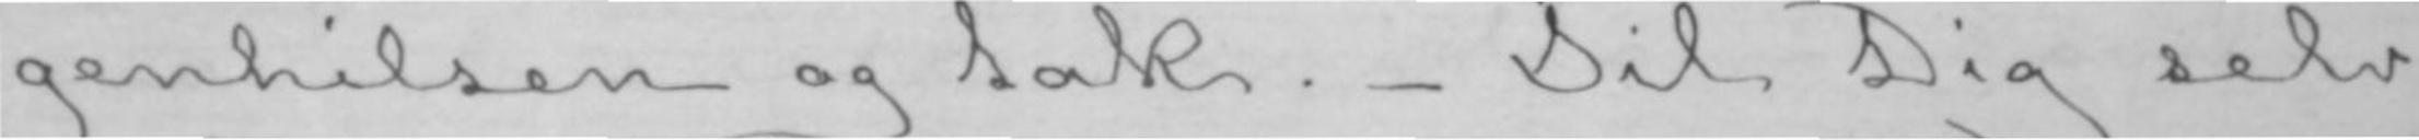genhilsen og tak.- Til Dig selv
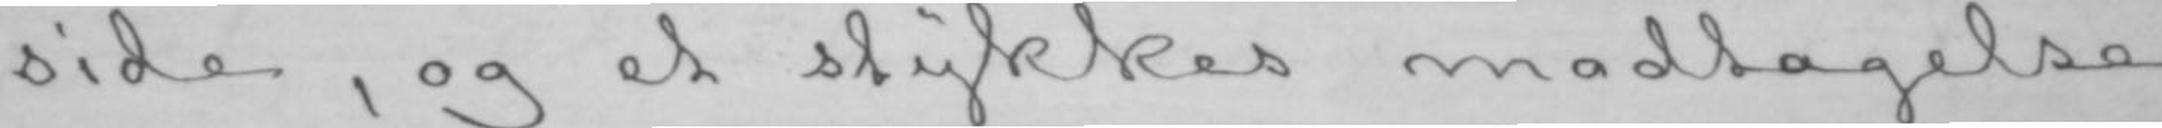side, og et stykkes modtagelse
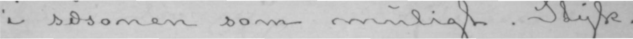i sæsonen som muligt. Styk-
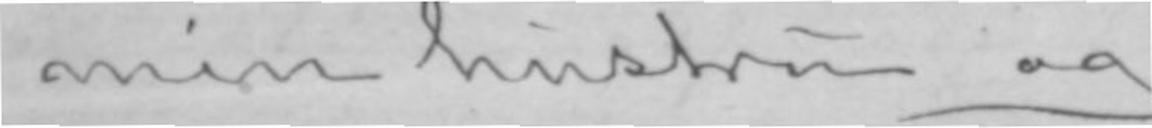min hustru og
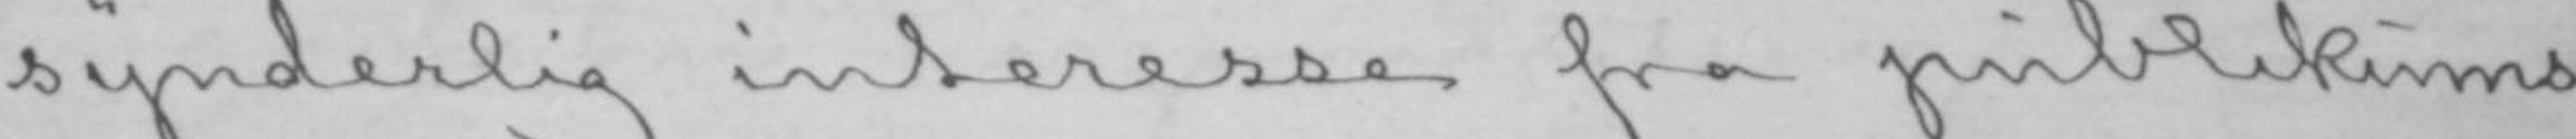synderlig interesse fra publikums
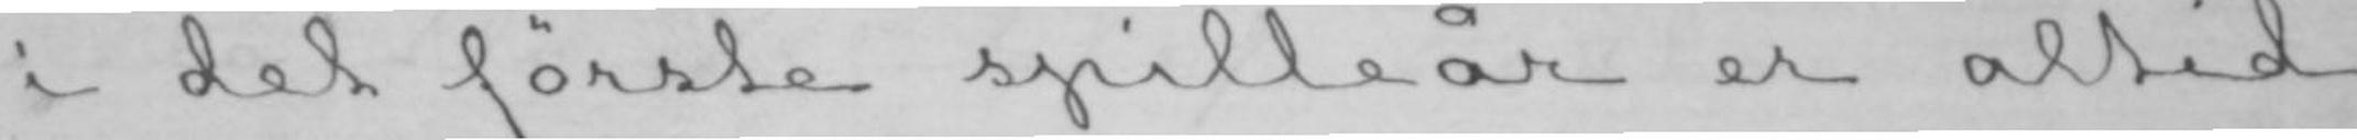i det første spilleår er altid
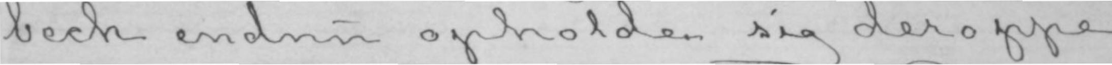bech endnu opholde sig deroppe,
